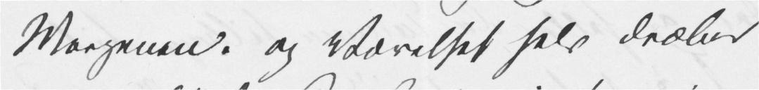Morgenen. og Værelset selv dræber
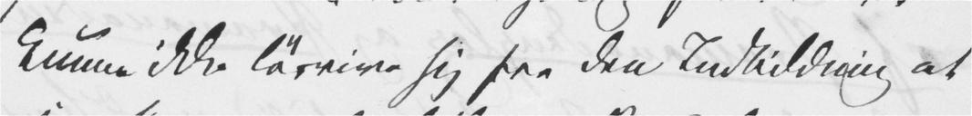kunne ikke løsrive sig fra den Indbildning at
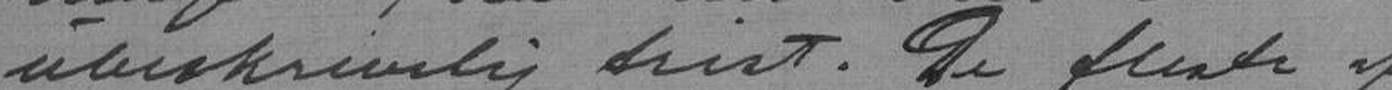ubeskrivelig trist. De fleste af
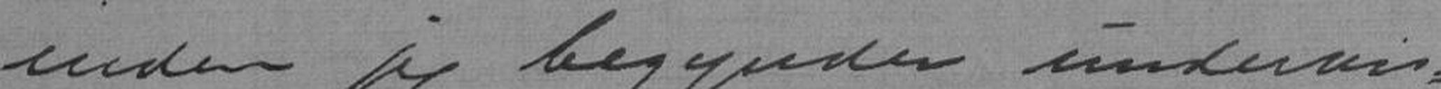inden jeg begynder undervis-
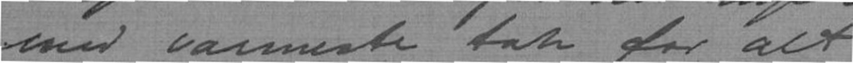med varmeste tak for alt
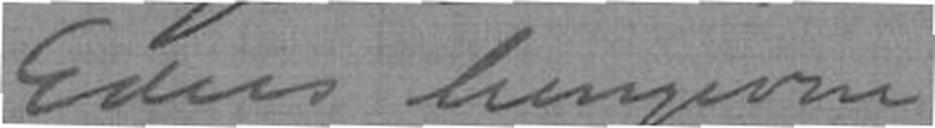Eders hengivne
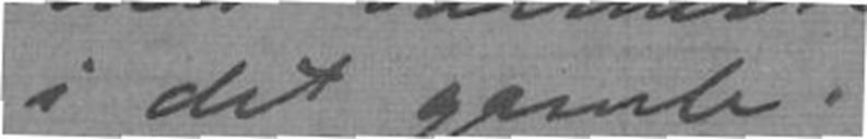i det gamle.
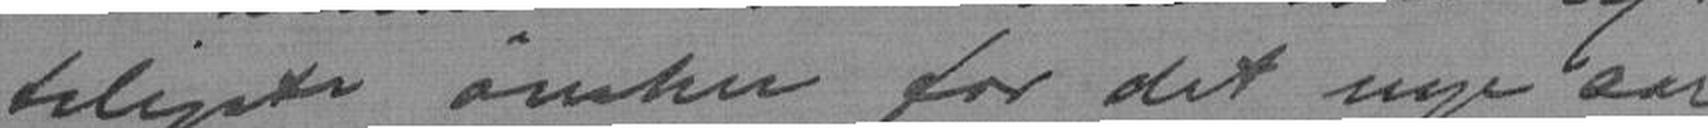teligste ønsker for det nye aar
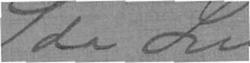Ida Lie
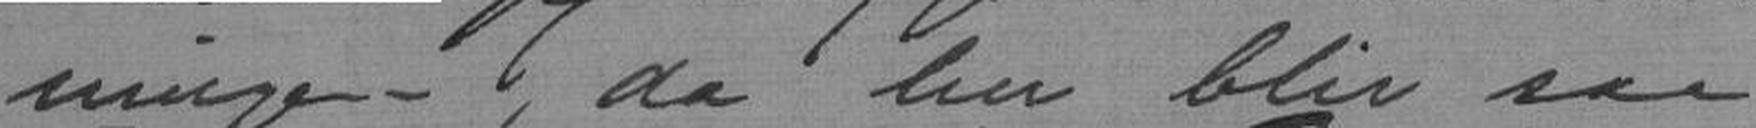ningen - , da her blir saa
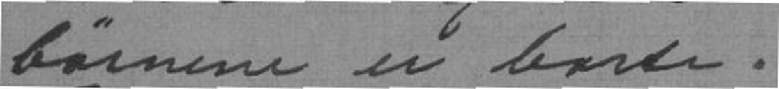børnen er borte.
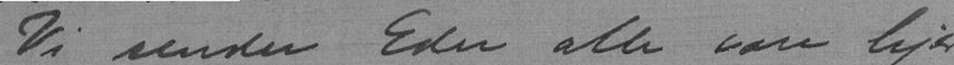Vi sender Eder alle vore hjær-
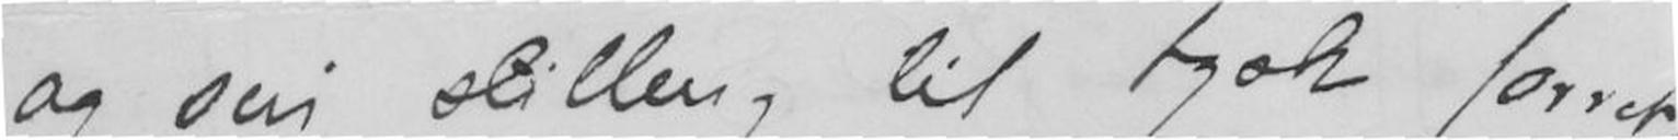og sin stilling til tysk forret-
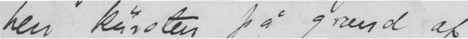herr Kursten på grund af
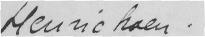Henrichsen.
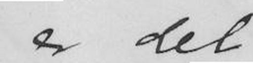er det
